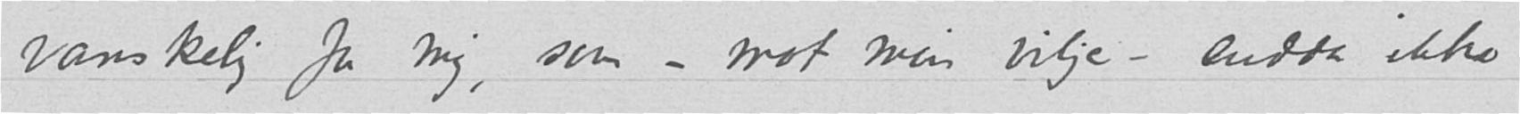vanskelig for mig, som – mot min vilje - endda ikke
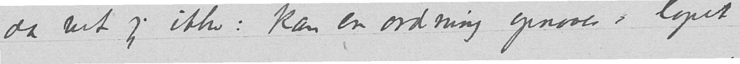da vet jeg ikke: kan en ordning opnaaes i løpet
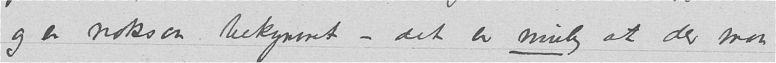og er noksaa bekymret – det er mulig at der maa
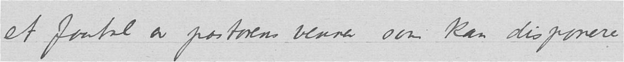et faatal av pastorens venner som kan disponere
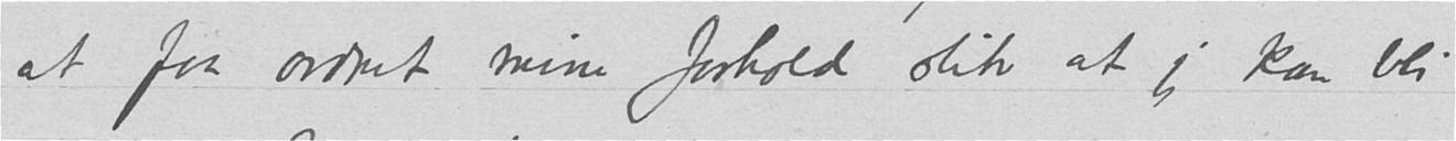at faa ordnet mine forhold slik at jeg kan bli
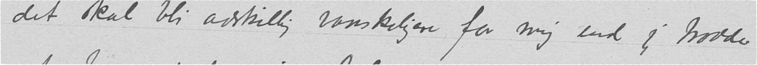det skal bli adskillig vanskeligere for mig end jeg trodde
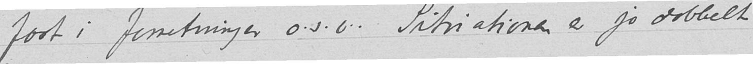fast i forretninger o.s.v.  Situationen er jo dobbelt
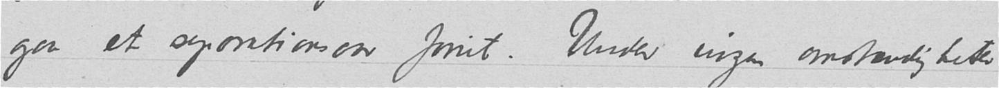gaa et separationsaar forut. Under ingen omstændigheter
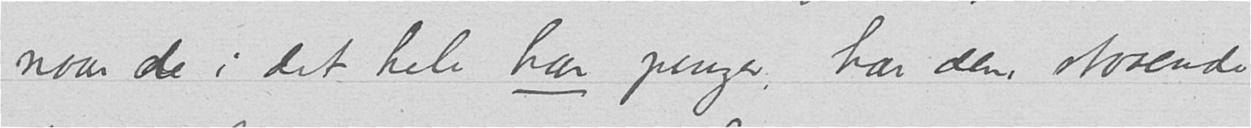naar de i det hele har penger, har dem staaende
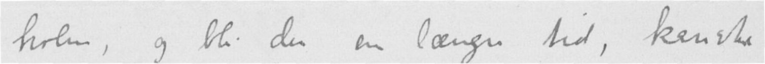holm, og bli der en længre tid, kanske
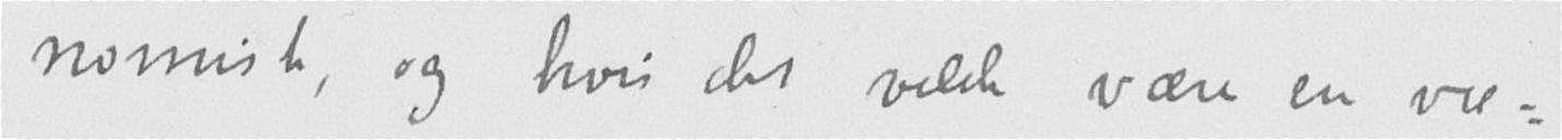nomisk, og hvis det vilde være en væ-
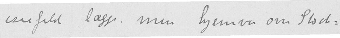isaafald lægge min hjemvei om Stock-
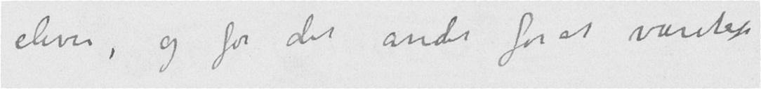elever, og for det andet for at væretes
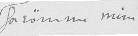forsømme mine
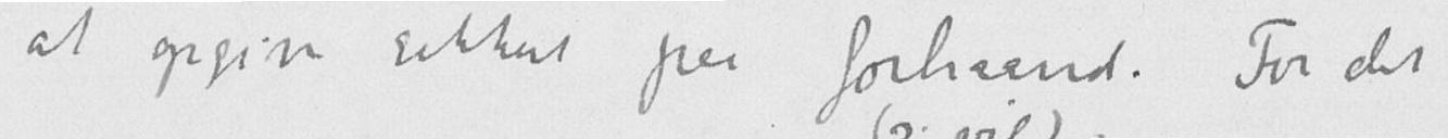at opgive sikkert paa forhaand. For det
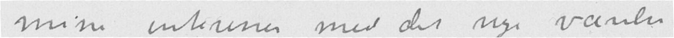mine interesser med det nye vænler
